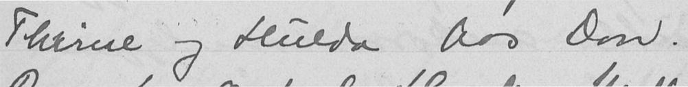Thrine og Hulda hos Don.
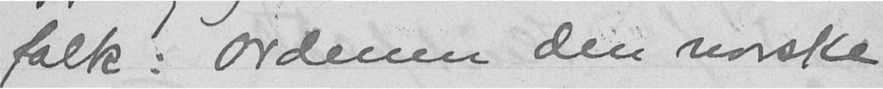folk: Ordenen den norske
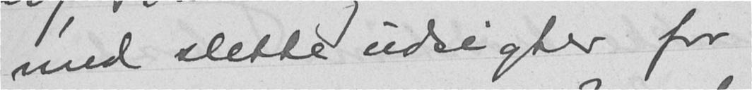med slette udsigter for
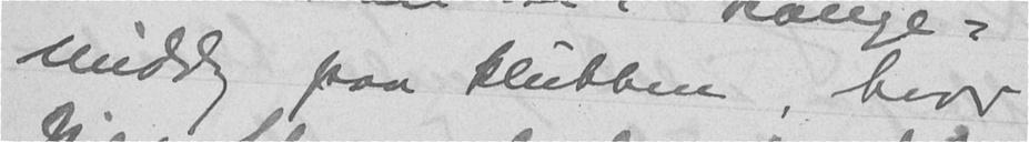middag paa klubben, hvor
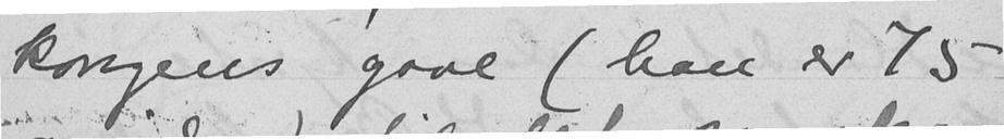kongens gave (han er 75
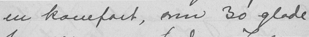en kanefart, som 30 glade
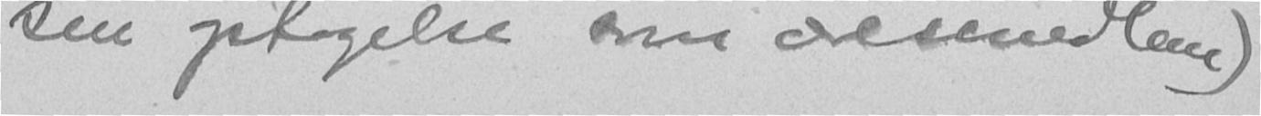sin optagelse som æresmedlem)
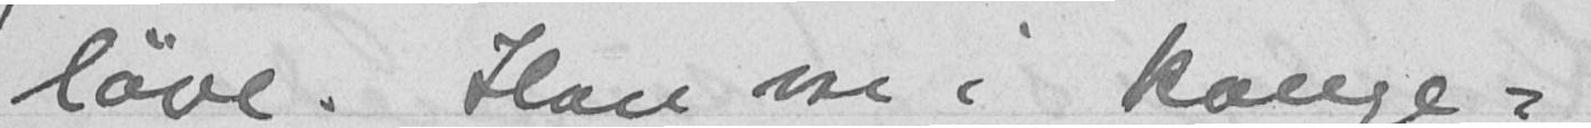løve. Han var i konge-
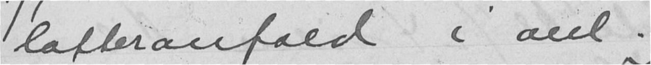latteranfald i anl.
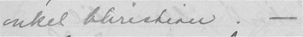onkel Christian. -
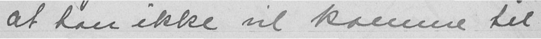at han ikke vil komme til
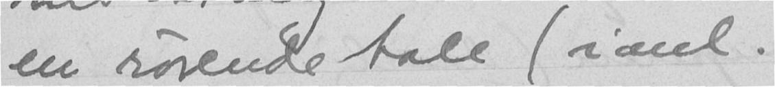en rørende tale (i anl.
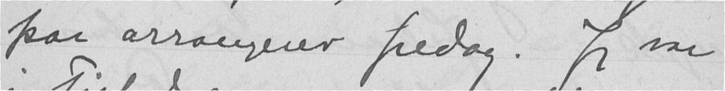par arrangerer fredag. Jeg var
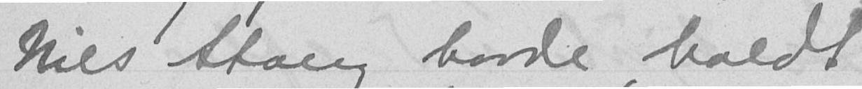Nils Stang havde holdt
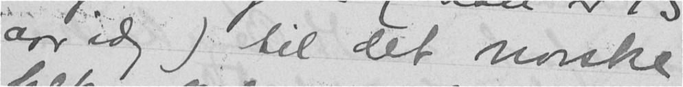aar idag) til det norske
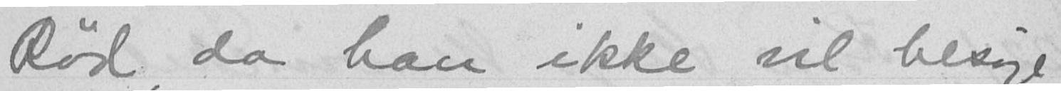Rød, da han ikke vil besøge
